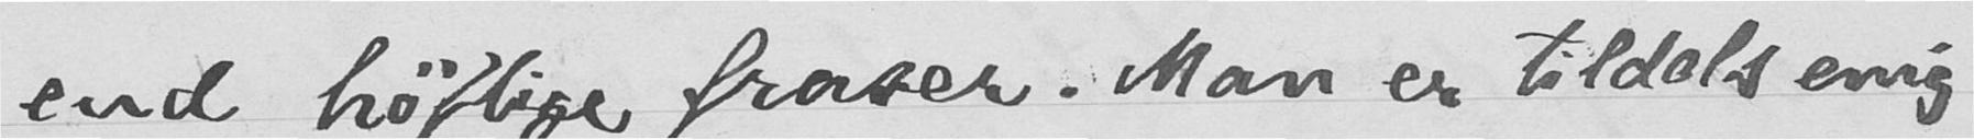end høflige fraser. Man er tildels enig
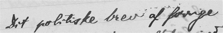Dit politiske brev af forrige
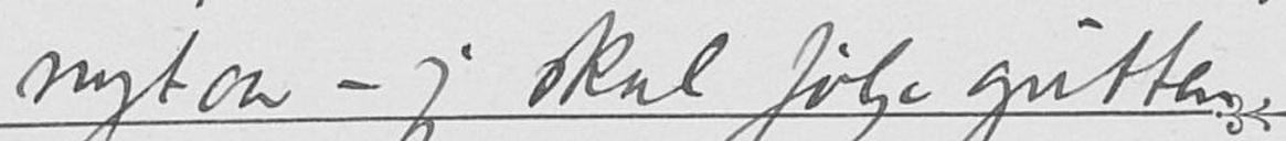nytaar – jeg skal følge gutten
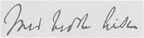Med bedste hilsen
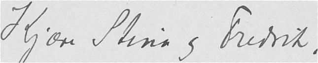Kjære Stina og Fredrik,
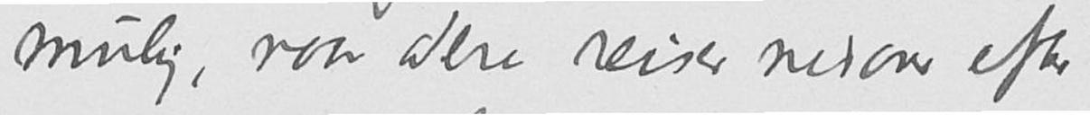mulig, naar dere reiser nedover efter
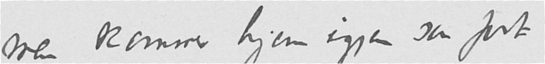men kommer hjem igjen saa fort
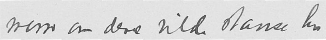morro om dere vilde stanse her
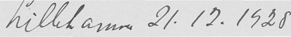Lillehammer 21.12.1928
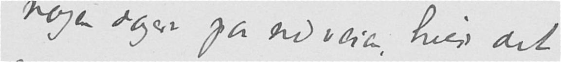nogen dager paa nedveien, hvis det
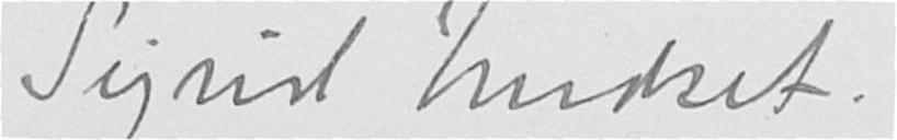Sigrid Undset.
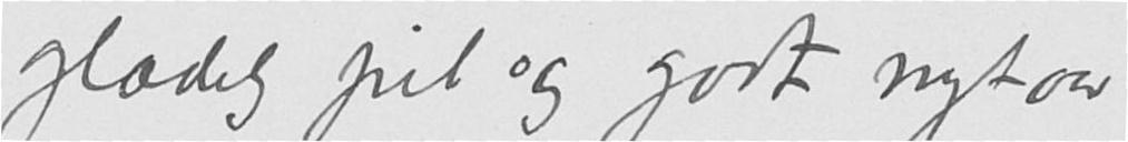glædelig jul og godt nytaar
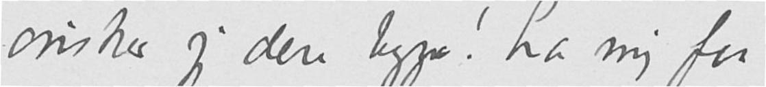ønsker ieg dere begge! La mig faa
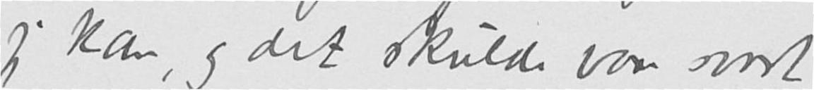jeg kan, og det skulde være svært
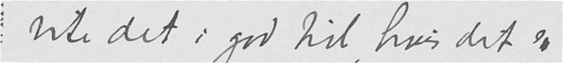vite det i god tid hvis det er
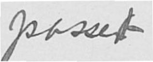passet.
